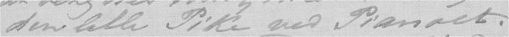den lille Pike ved Pianoet.
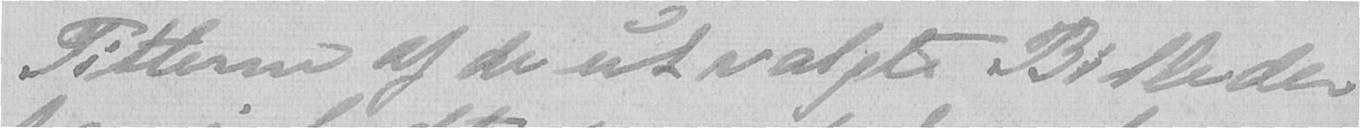Titlerne af de utvalgte Billeder
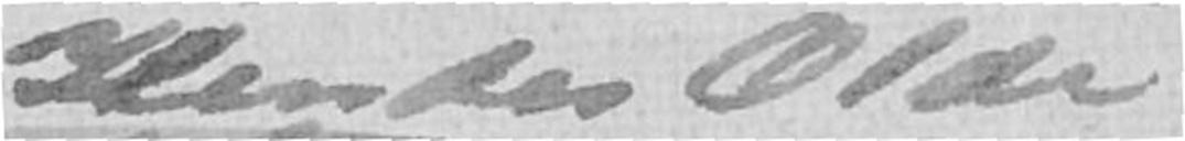Hendes Olde
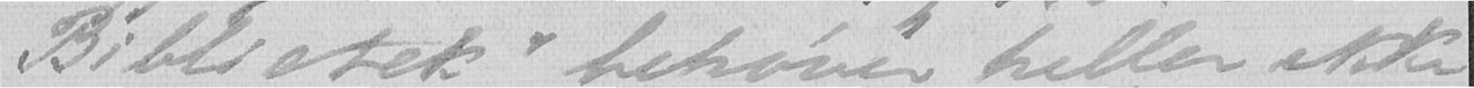Bibliotek" behøver heller ikke
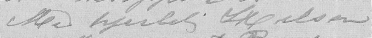Med hjertelig Hilsen
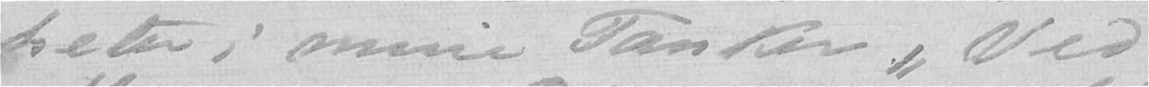heter i mine Tanker "Ved
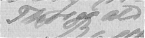Thorvald
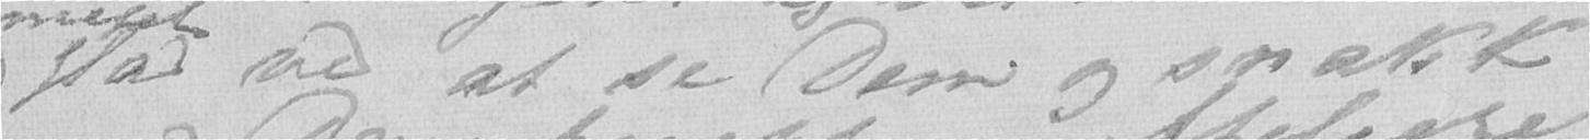glad ved at se Dem og snakke
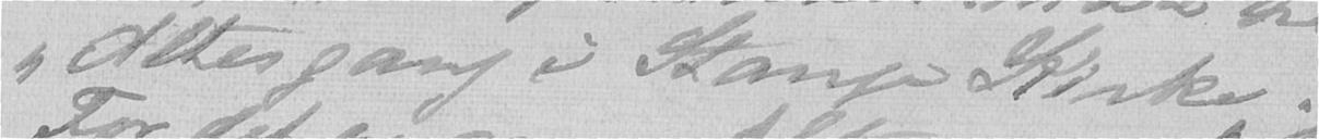"Altergang i Stange Kirke".
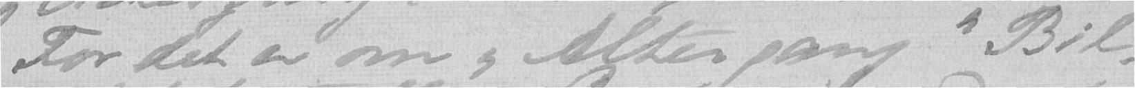For det er om "Altergang" Bil-
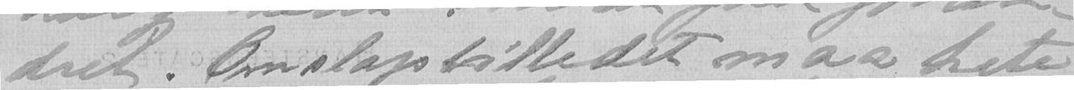dret. Omslagsbilledet maa hete
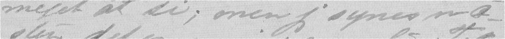meget at si; men jeg synes næ-
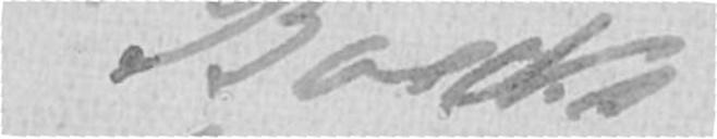Boecks
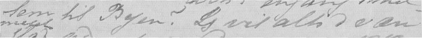lem til Byen? Jeg vil altid være
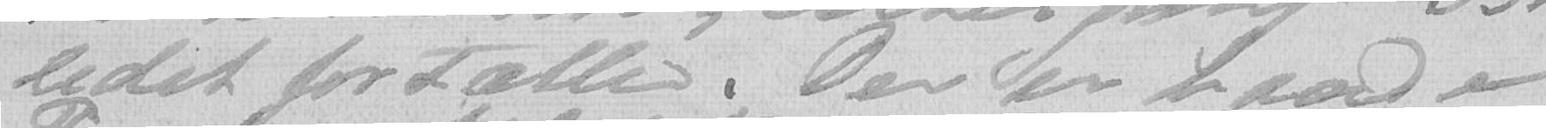ledet fortæller. Der er baade
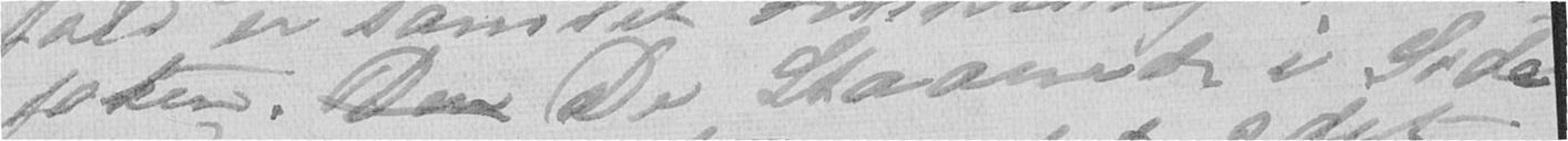foten. Den De Staaende i Side-
